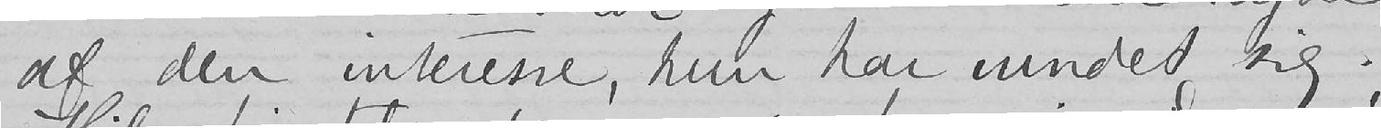af den interesse, hun har vundet sig.
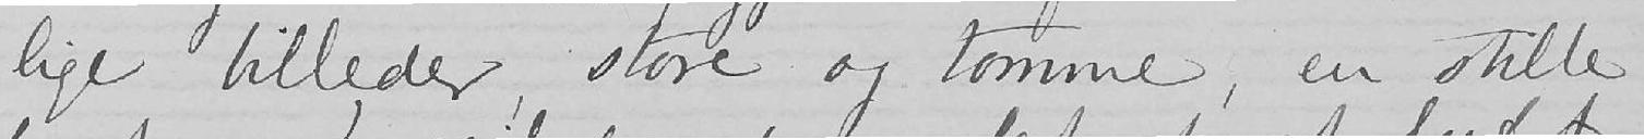lige billeder, store og tomme, en stille
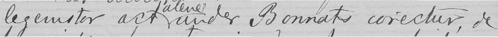legemstor act under Bonnats corectur, de
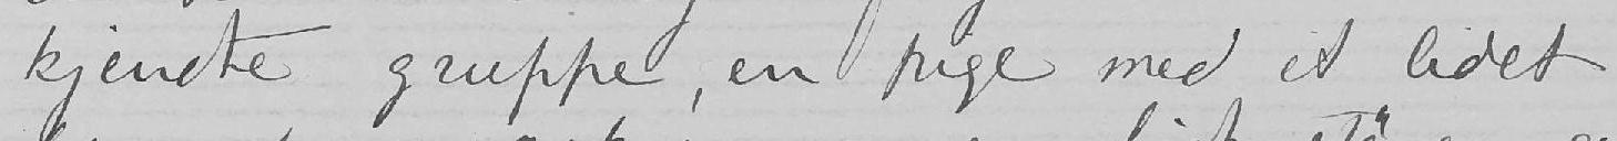kjendte gruppe, en pige med et lidet
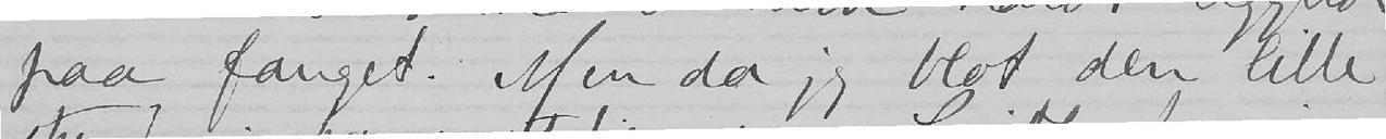paa fanget. Men da jeg blot den lille
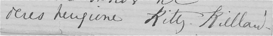deres hengivne Kitty Kielland.
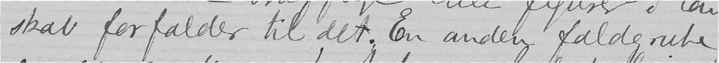skab forfalder til det. En anden faldgrube
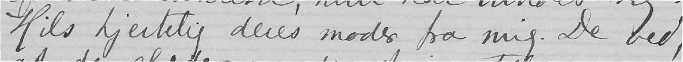Hils hjertelig deres moder fra mig. De ved,
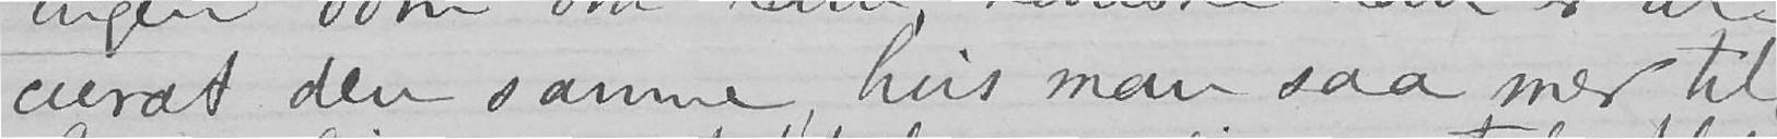curat den samme, hvis man saa mer til
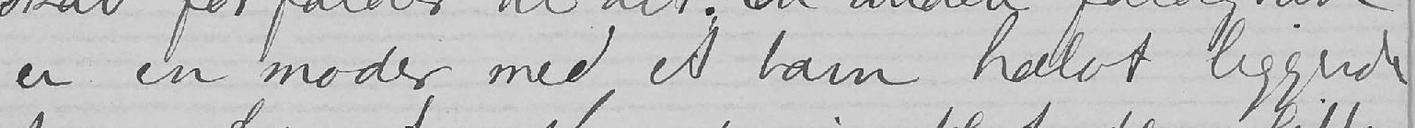er en moder med et barn halvt liggende
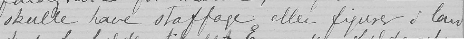skulle have staffage eller figurer i land-
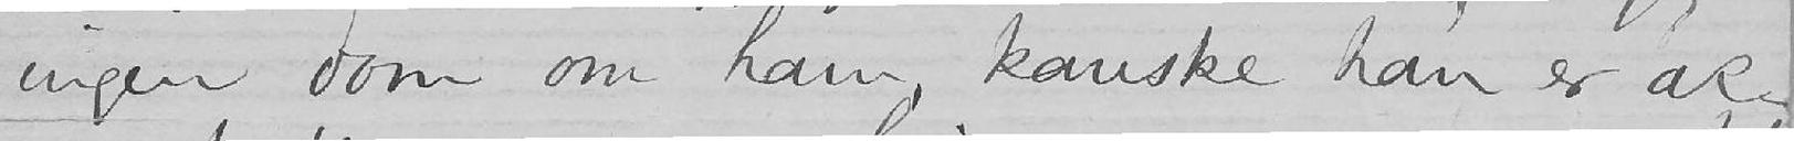ingen dom om ham, kanske han er ac-
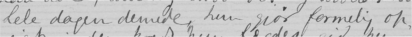hele dagen dernede, hun gjør formelig op-
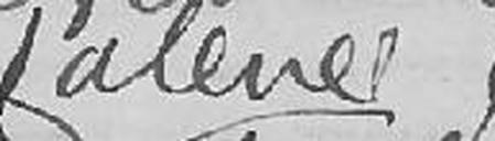alene
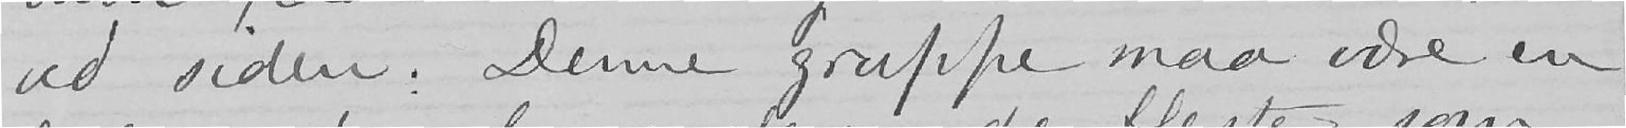ved siden. Denne gruppe maa være en
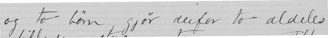og to børn, gjør derfor to aldeles
